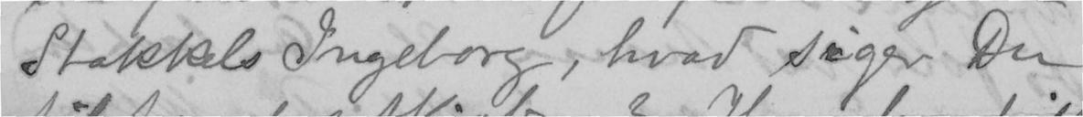Stakkels Ingeborg, hvad siger Du
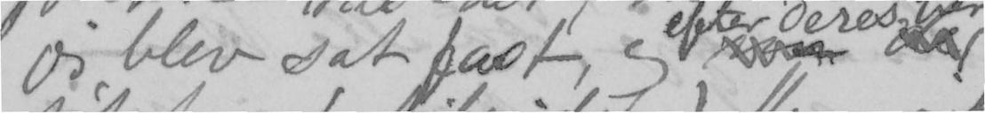jeg blev sat fast, og m de
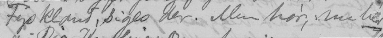Tyskland, siges der. Men hør, nu ber
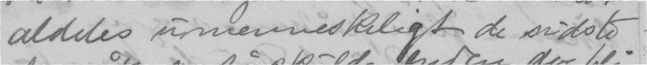aldeles umenneskeligt de sidste
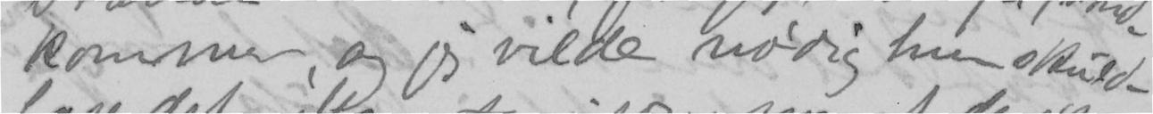kommer, og jeg vilde nødig hun skulde
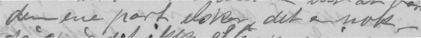den ene part elsker, det er nok -
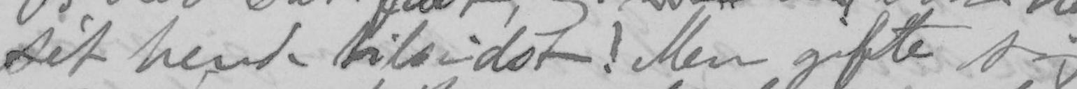sét hende tilsidst! Men gifte sig
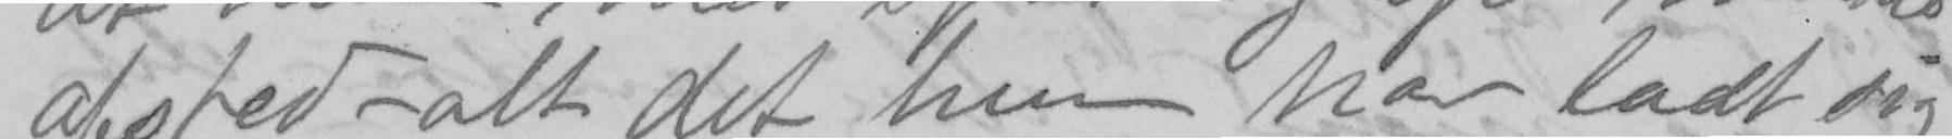afsted - alt det hun har ladt sig
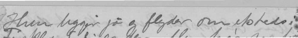Hun ligger jo og flyder om etsteds i
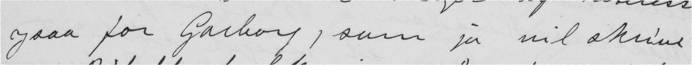ogsaa for Garborg, som jo vil skrive
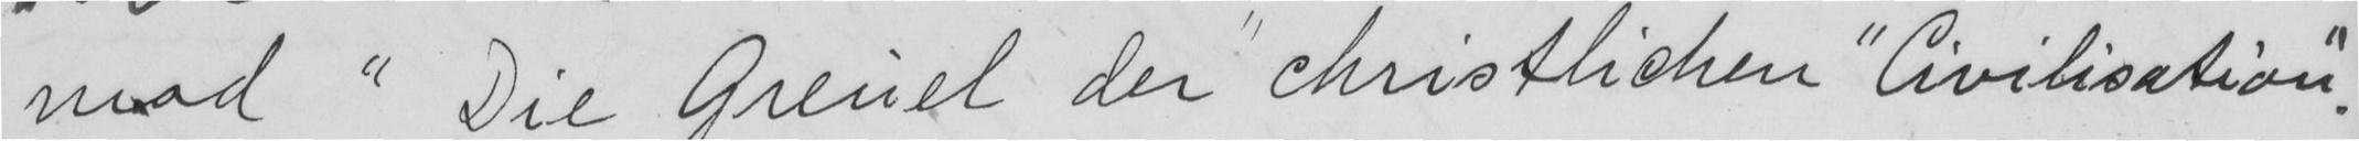mod "Die Greuel der" christlichen "Civilisation".
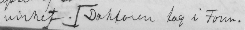virket. Doktoren tog i Form.
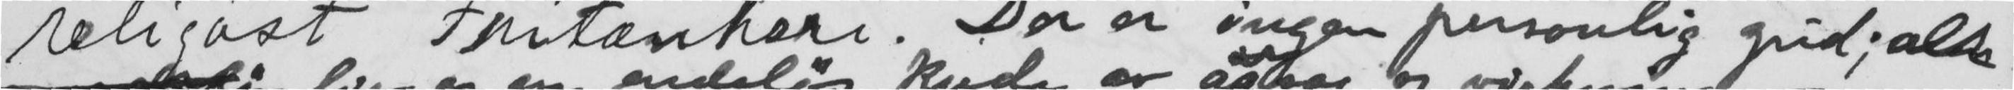religøst Fritænkeri. Der er ingen personlig gud; alle
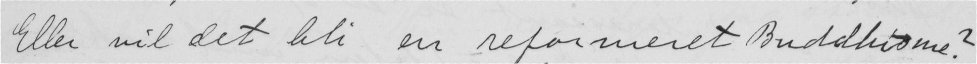Eller vil det bli en reformeret Buddhisme?
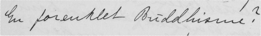En forenklet Buddhisme?
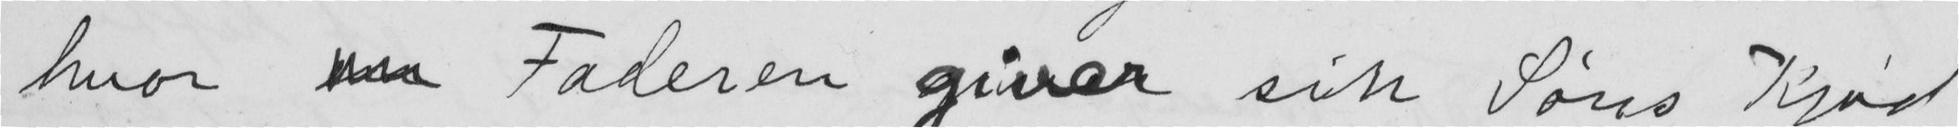hvor  Faderen giver sin Søns Kjød
